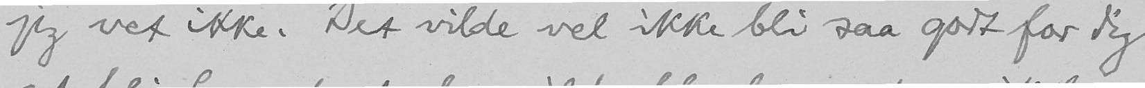jeg vet ikke. Det vilde vel ikke bli saa godt for dig
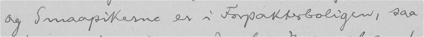og Smaapikerne er i Forpakterboligen, saa
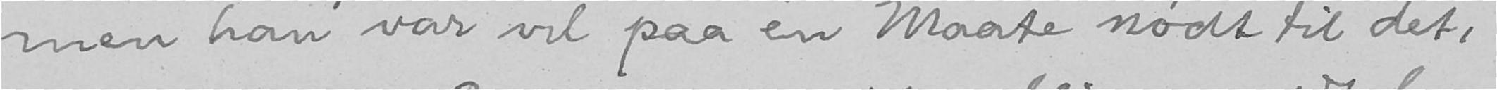men han var vel paa en Maate nødt til det,
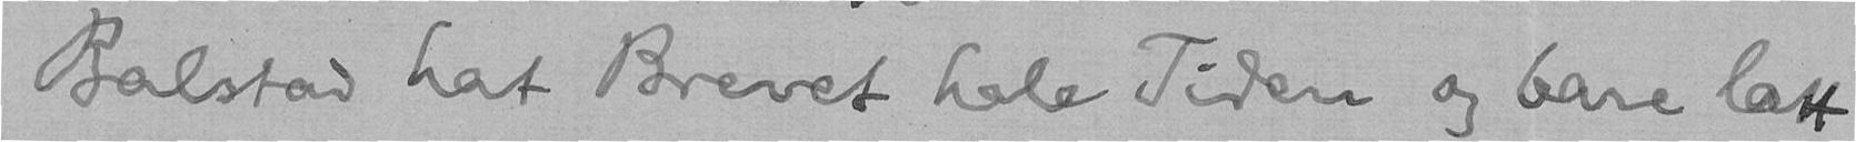Balstad hat Brevet hele Tiden og bare latt
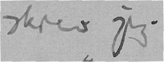skrev jeg.
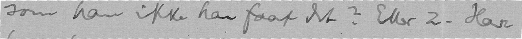som han ikke har faat det? Eller 2. Har
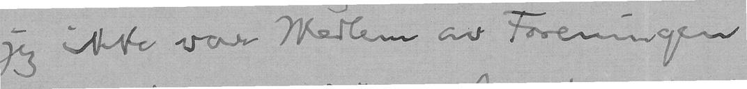jeg ikke var Medlem av Foreningen
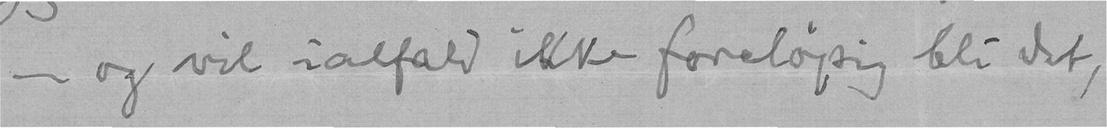- og vil ialfald ikke foreløpig bli det,
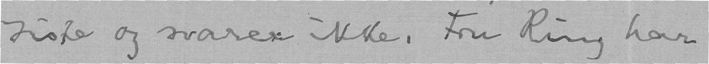siste og svarer ikke. Fru Ring har
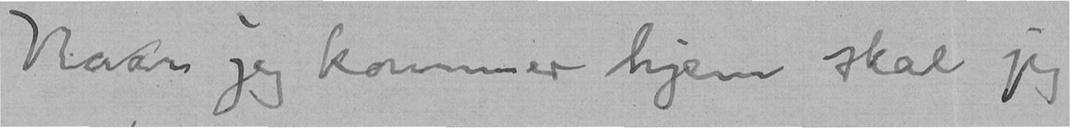Naar jeg kommer hjem skal jeg
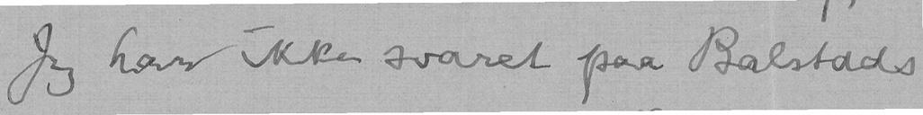Jeg har ikke svaret paa Balstads
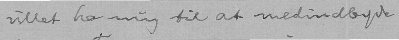villet ha mig til at medindbyde
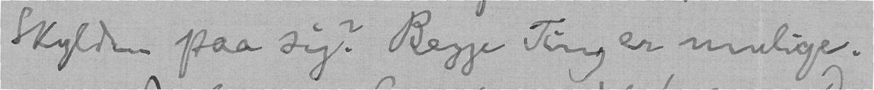Skylden paa sig? Begge Ting er mulige.
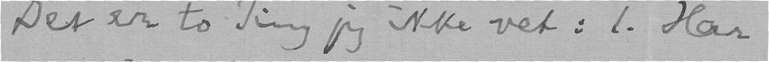Det er to Ting jeg ikke vet: 1. Har
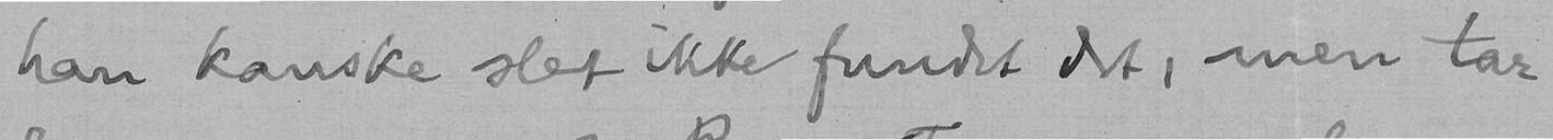han kanske slet ikke fundet det, men tar
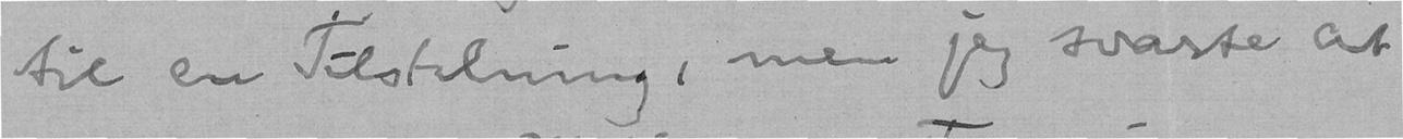til en Tilstelning, men jeg svarte at
